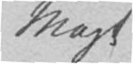Magt
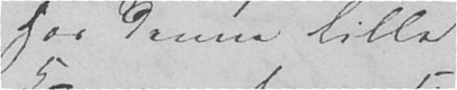hos denne lille
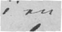i en
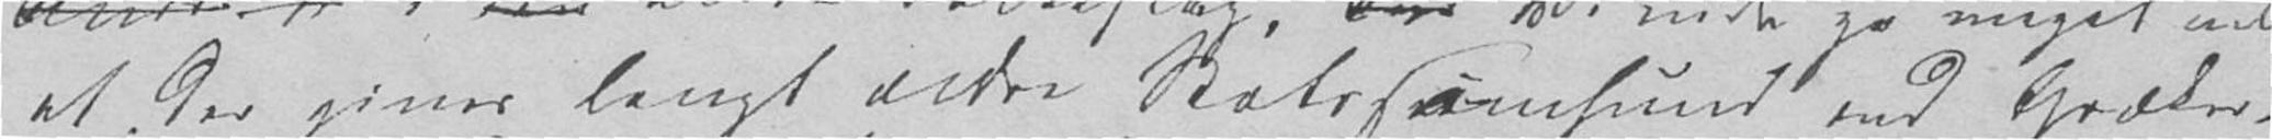at der gives langt ældre Statssamfund end Græker-
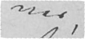nes,
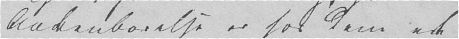Aabenbarelse er hos dem et
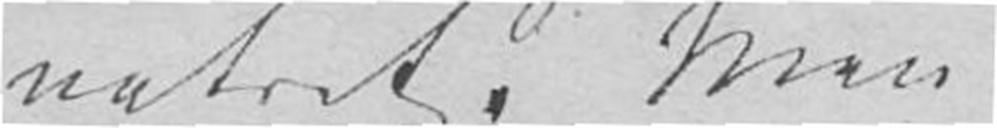vatret. Men
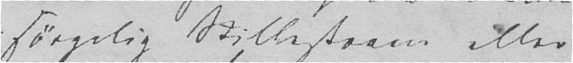sørgelig Stillestaaen eller
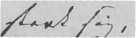strak sig,
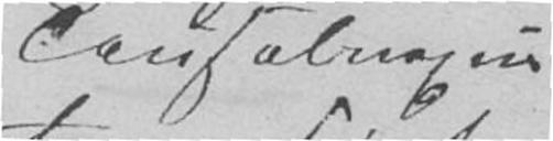Causalvoxen
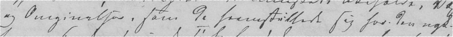og Omgivelser, som de fremstillede sig for den nøk-
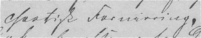chaotisk Forvirring.
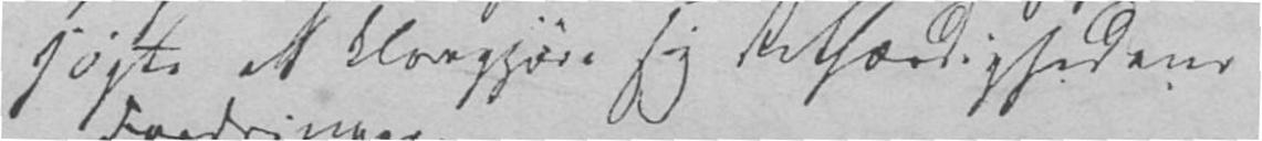søgte at klargjøre sig Retfærdighedens
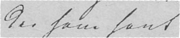der have havt
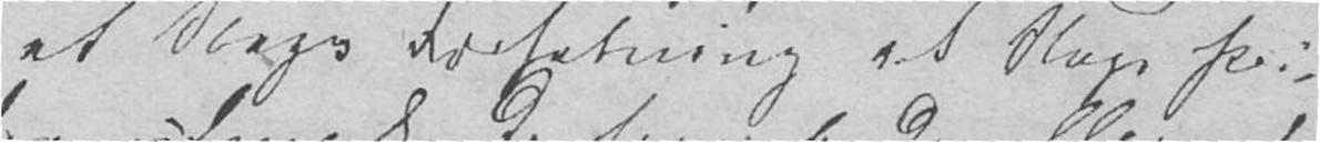et slags Forfatning et Slags Pri-
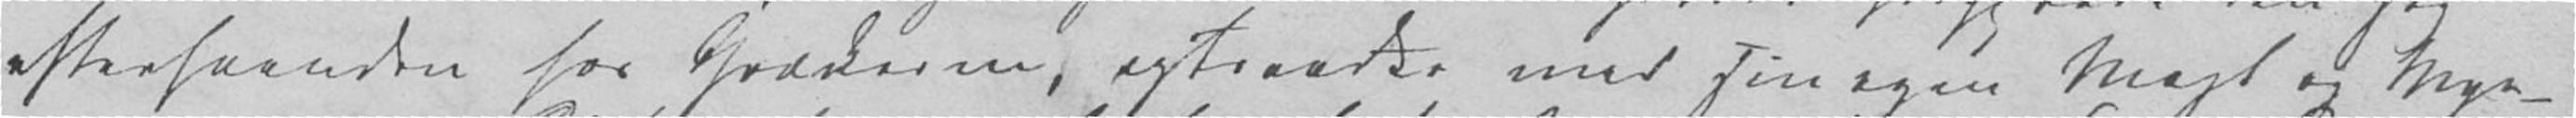efterhaanden hos Grækerne, optraadte med sin egen Magt og Myn-
